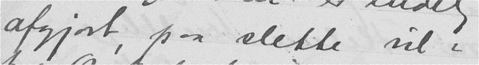afgjort, paa slette vil i
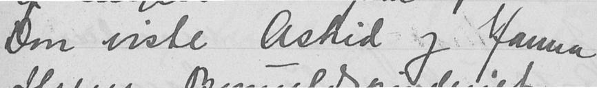Don viste Astrid og Janna
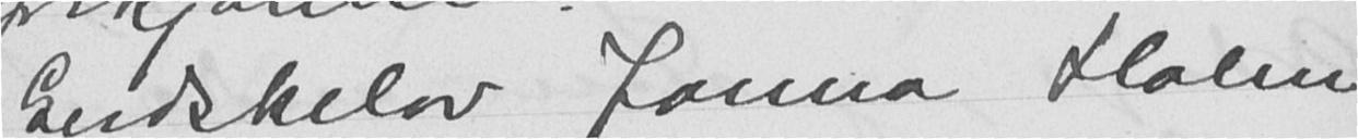Gudskelov Janna Holm
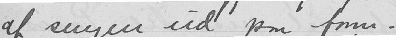af sengen ud paa form.
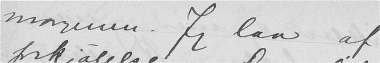morgenen. Jeg laa af
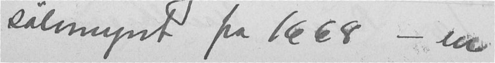sølvmynt fra 1668 - en
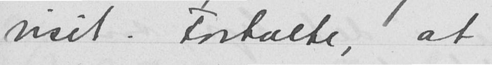visit. Fortalte, at
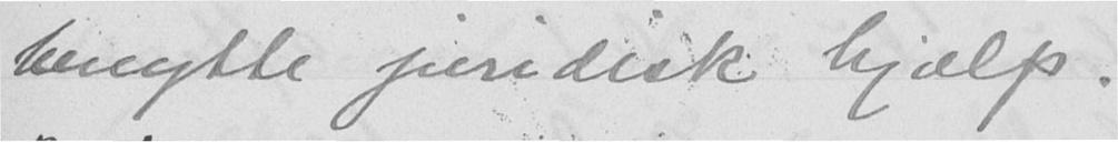benytte juridisk hjælp.
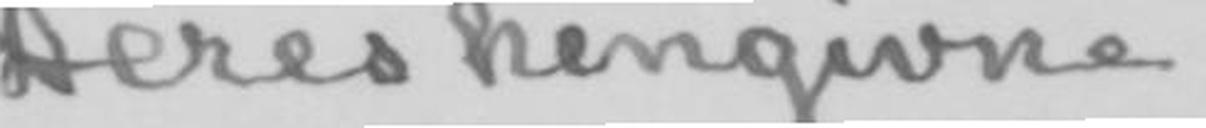Deres hengivne
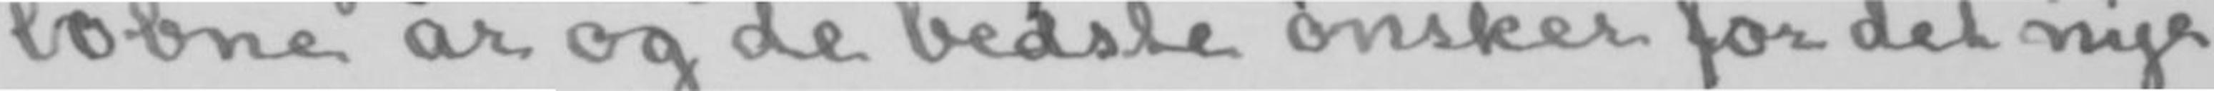løbne år og de bedste ønsker for det nye.
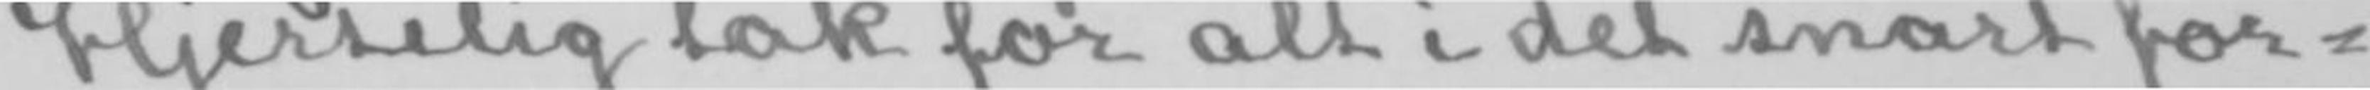Hjertelig tak for alt i det snart for-
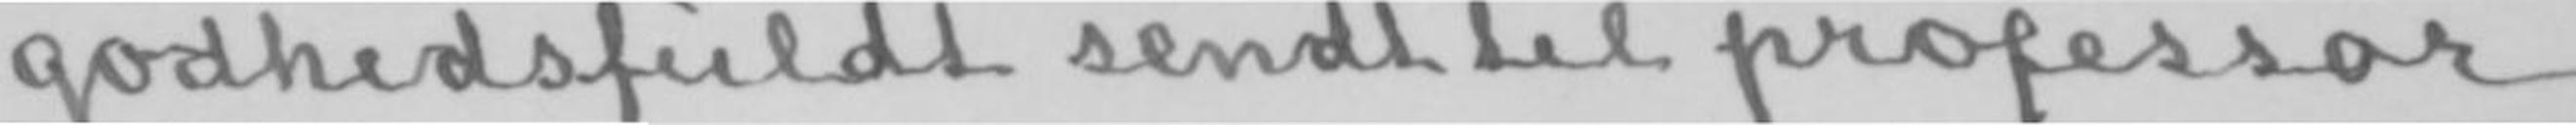godhedsfuldt sendt til professor
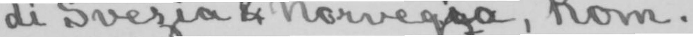di Svezia & Norvegia, Rom.
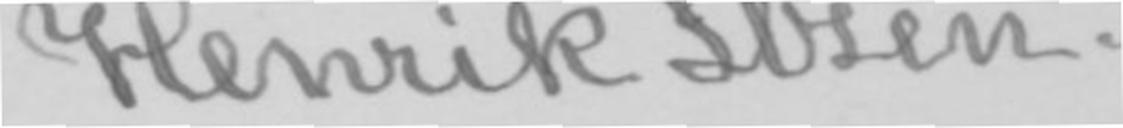Henrik Ibsen.
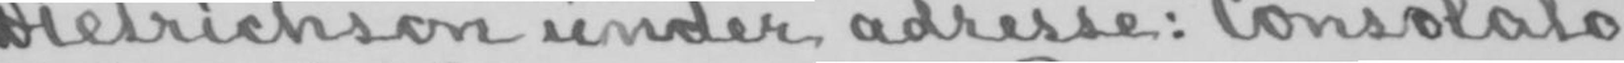Dietrichson under adresse: Consolato
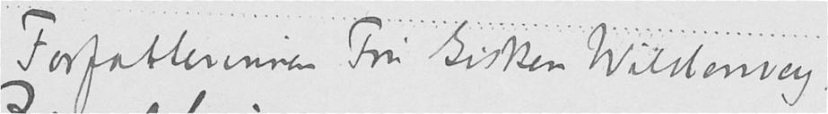Forfatterinnen Fru Gisken Wildenvey,
